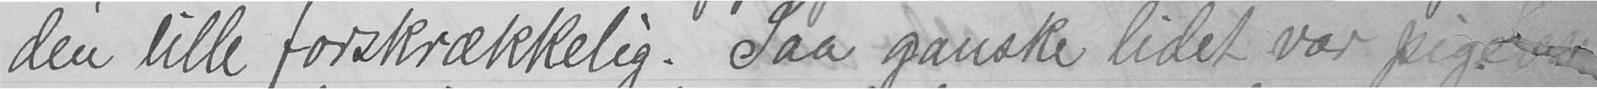den lille forskrækkelig. Saa ganske lidet var pigebar-
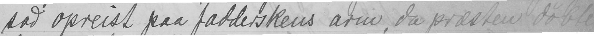sad opreist paa fadderskens arm, da præsten døbte
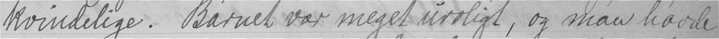kvidelige. Barnet var meget uroligt, og man havde
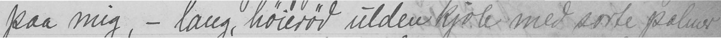paa mig, - lang, høierød ulden kjole med sorte palmer
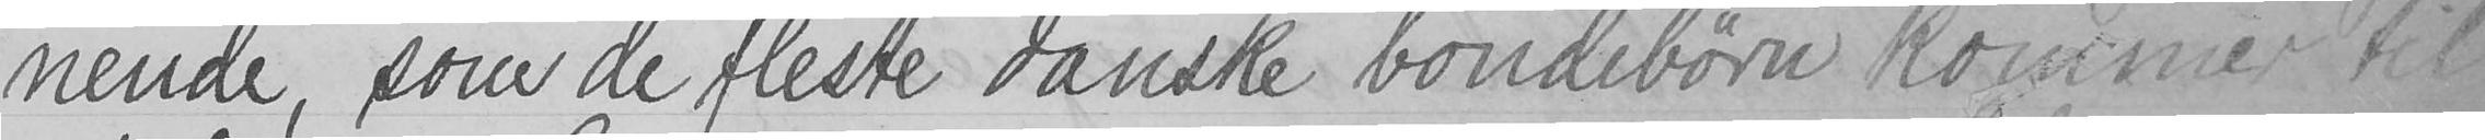nende, som de fleste danske bondebørn kommer til
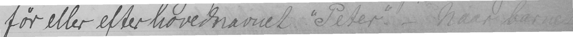før eller efter hovednavnet "Peter". - Naar barnene
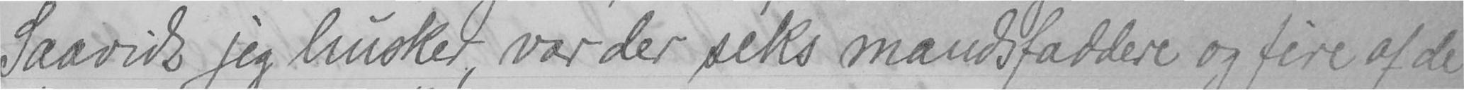Saavidt jeg husker, var der seks mandsfaddere og fire af de
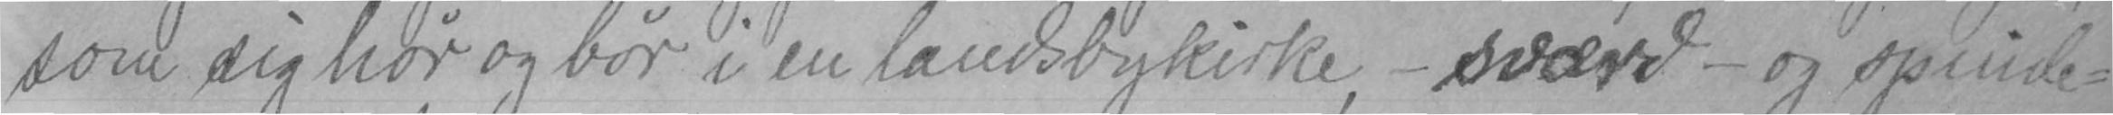som sig hør og bør i en landsbykirke, - sværd- og spinde-
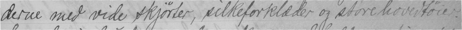derne med vide skjørter, silkeforklæder og store hovedtøier.
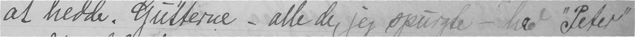at hedde. Gutterne - alle de, jeg spurgte - hed "Peter"
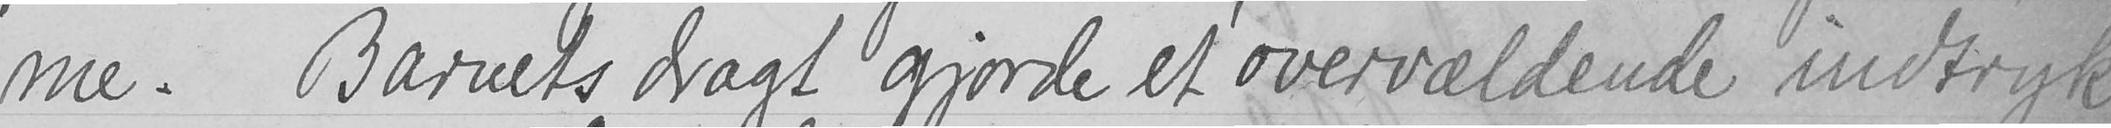me. Barnets dragt gjorde et overvældende indtryk
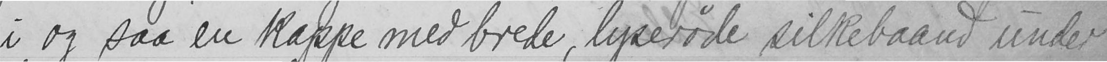i og saa en kappe med brede, lyserøde silkebaand under
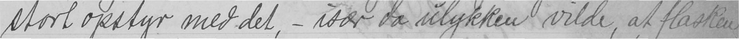stort opstyr med det, - især da ulykken vilde, at flasken
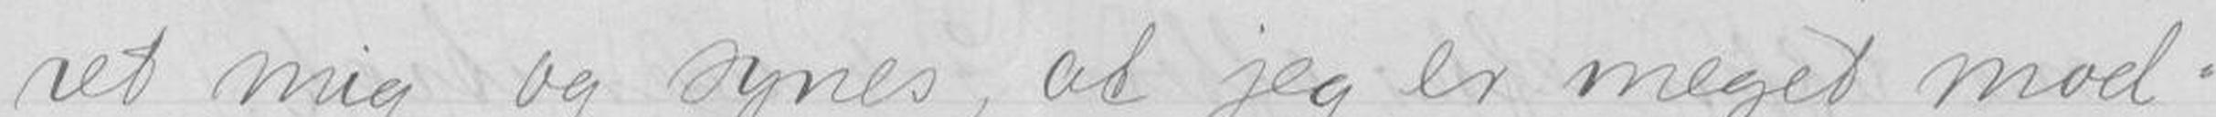ret mig og synes, at jeg er meget mod-
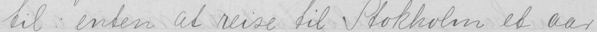til: enten at reise til Stokholm et aar
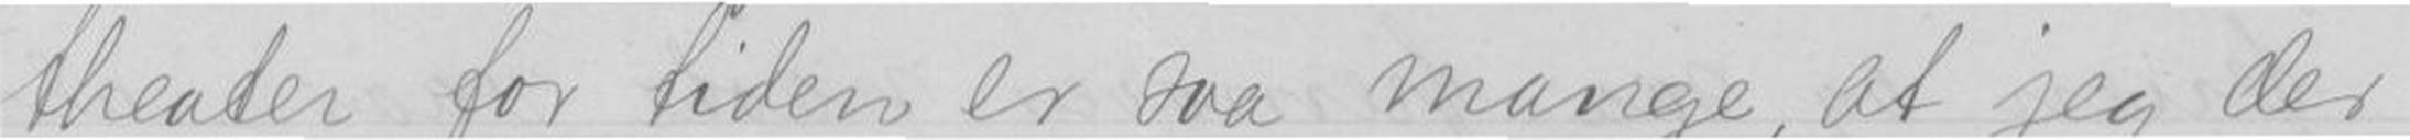theater for tiden er saa mange, at jeg der
